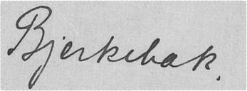Bjerkebæk,
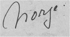Norge.
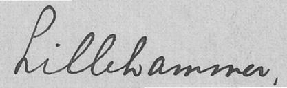Lillehammer,
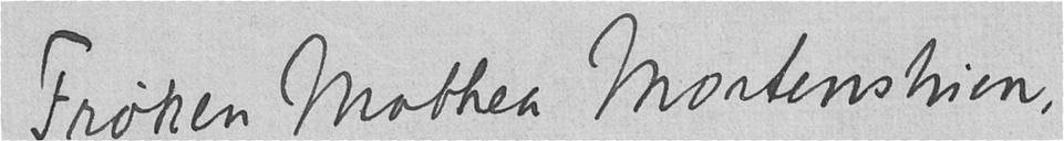Frøken Mathea Mortenstuen,
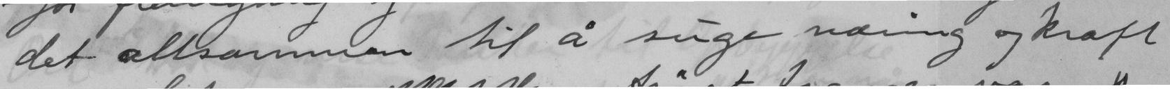det altsammen til å suge næring og kraft
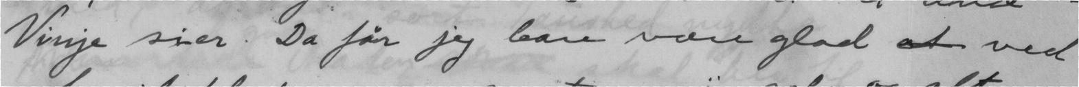Vinje sier. Da får jeg bare være glad at ved
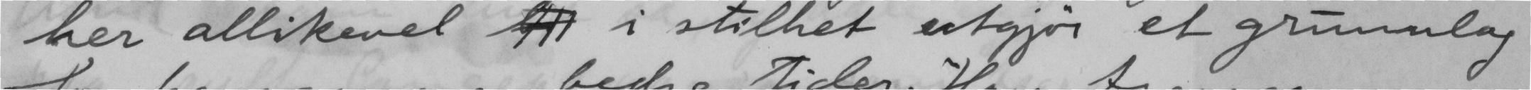her allikevel  i stilhet udgjør et grunnlag
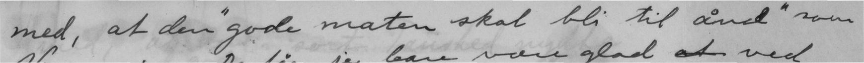med, at den "gode maten skal bli til ånd" som
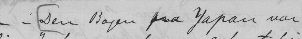- - Den Bogen fra Japan var
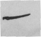-
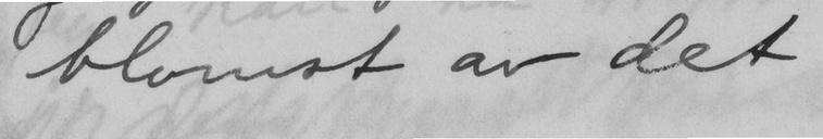blomst av det
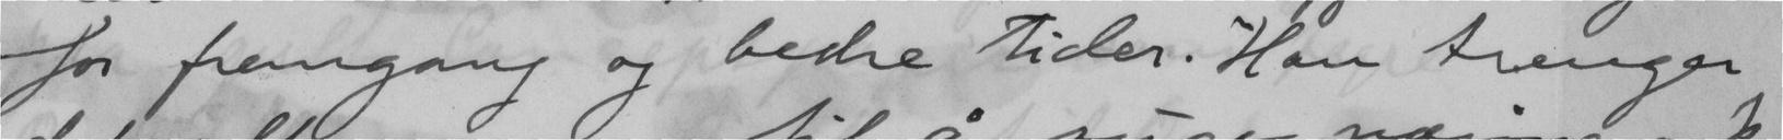for fremgang og bedre tider. Han trenger
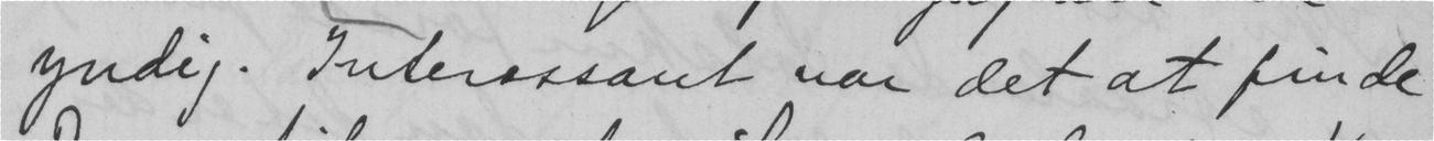yndig. Interessant var det at finde
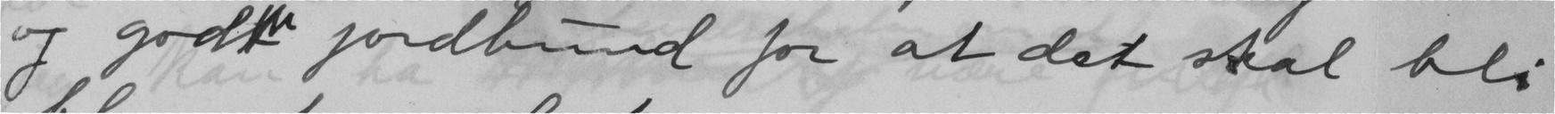og godt jordbund for at det skal bli
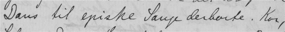Dans til episke Sange derborte. Kor,
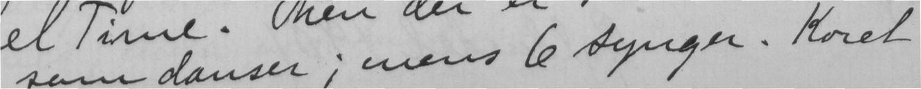som danser; mens 6 synger. Koret
